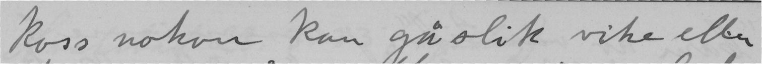koss nokon kan gå slik vike etter
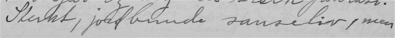Sterkt, jordbunde sanseliv, men
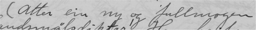(Atter ein ny og fullmogen
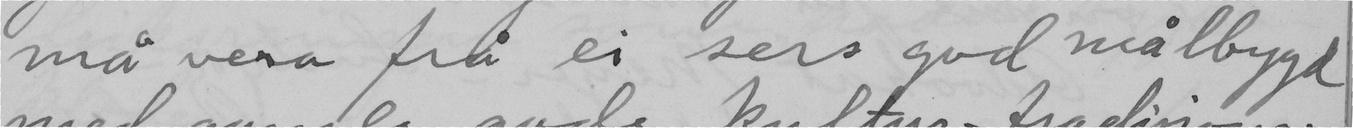må vera frå ei sers god målbygd
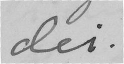dei.
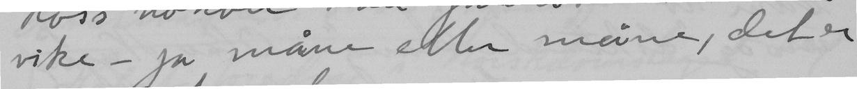vike – ja måne etter måne, det er
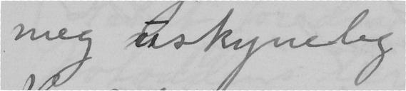meg uskyneleg.
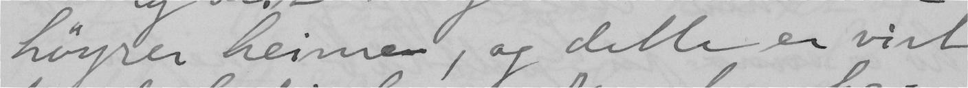höyrer heime, og dette er vist
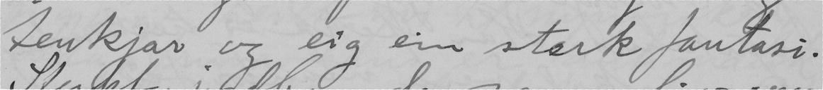tenkjar og eig ein stærk fantasi.
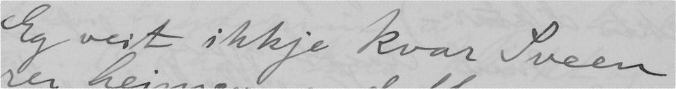Eg veit ikkje kvar Sveen
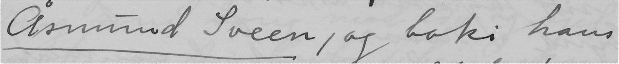Åsmund Sveen, og boki hans
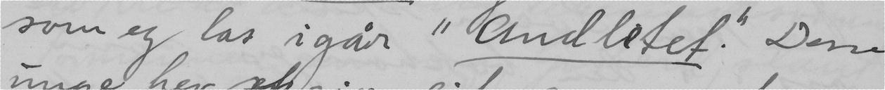som eg las igår "Andletet". Desse
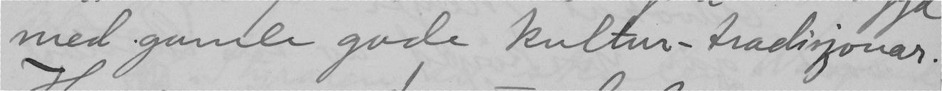med gamle gode kultur-tradisjonar.
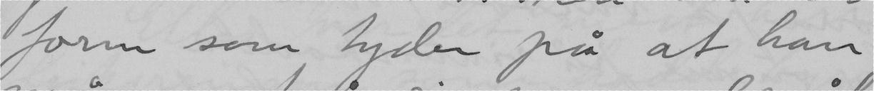form som tyder på at han
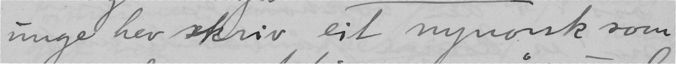unge hev skriv eit nynorsk som
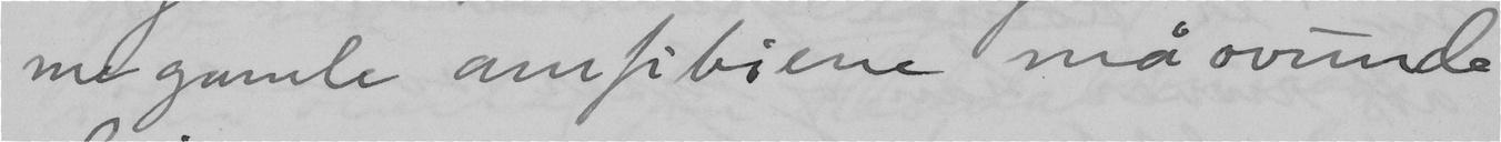me gamle amfibiene må ovunde
